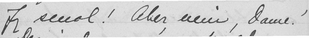Jeg smal! Aber, mein, Dame!
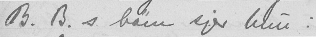B. B.s børn siger hun:
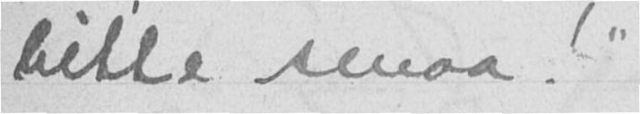bitte smaa!"
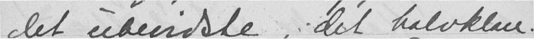det ubevidste, det halvklare.
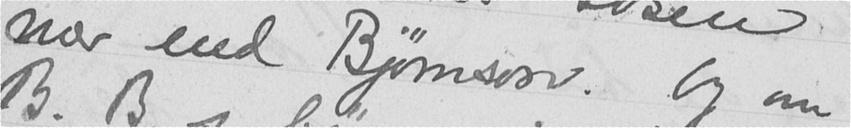mer end Bjørnson. Og om
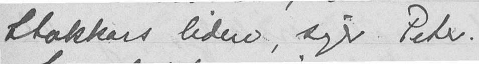Stakkars liden, siger Peter.
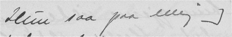Hun saa paa mig og
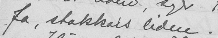Ja, stakkars liden.
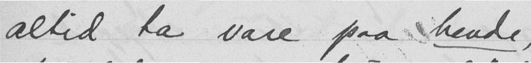altid ta vare paa hende,
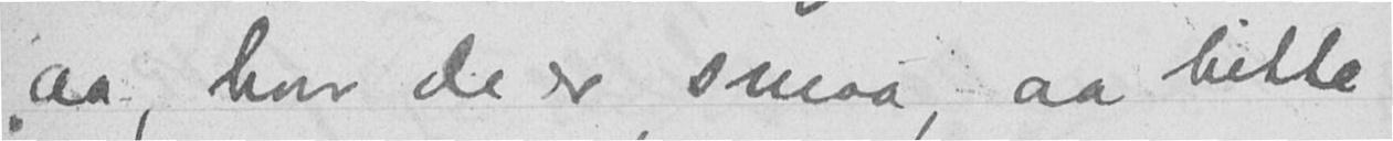"aa, hvor de er smaa, aa bitte
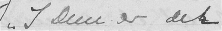"I Dem er det
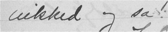nikked og sa:
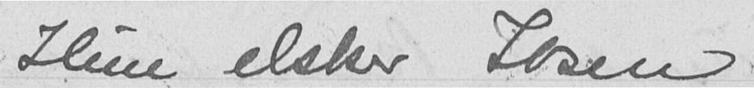Hun elsker Ibsen
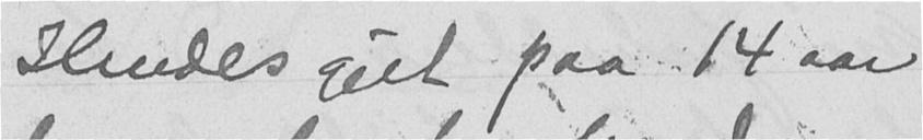Hendes gut paa 14 aar
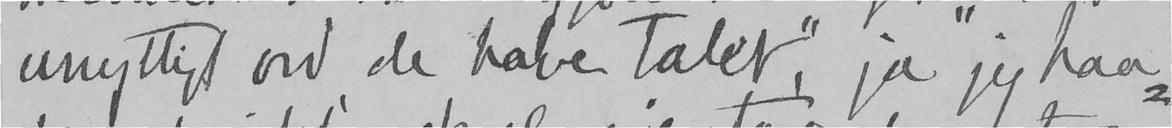unyttigt ord, de have talet", ja jeg haa-
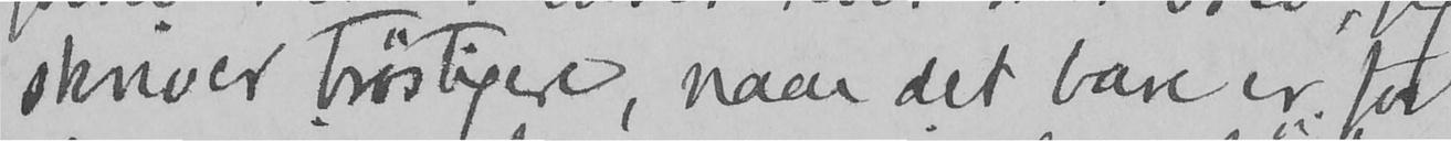skriver trøstigere, naar det bare er for
